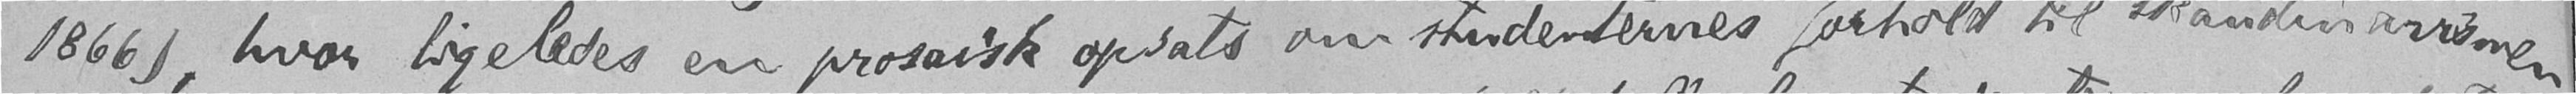1866), hvor ligeledes en prosaisk opsats om studenternes forhold til skandinavismen
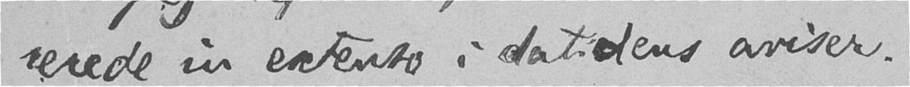rerede in extenso i datidens aviser.
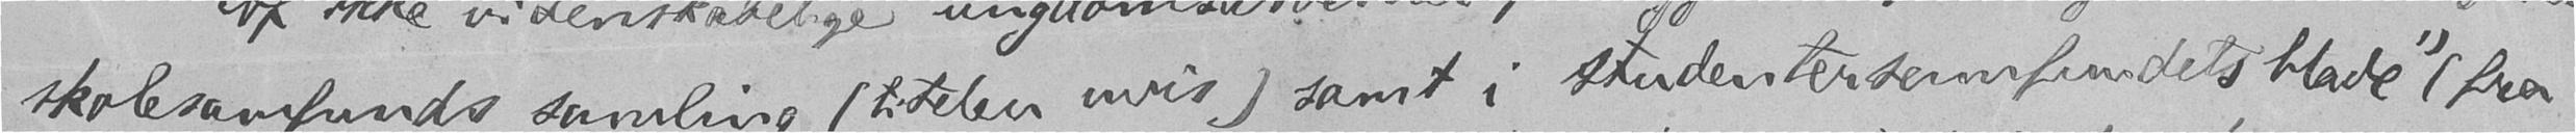skolesamfunds samling (titelen uvis) samt i studentersamfundets blade 1) (fra
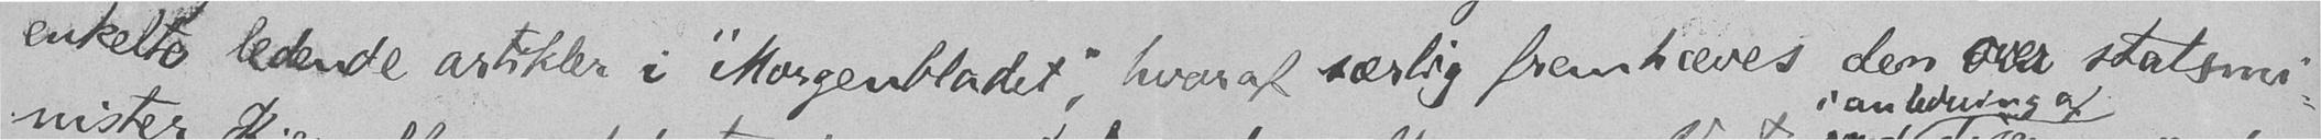enkelte ledende artikler i "Morgenbladet", hvoraf særlig fremhæves den over statsmi-
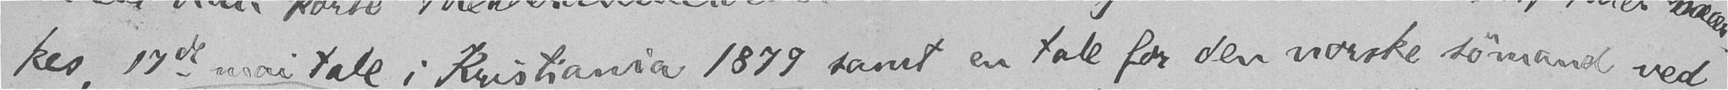kes, 17de mai tale i Kristiania 1879 samt en tale for den norske sømand ved
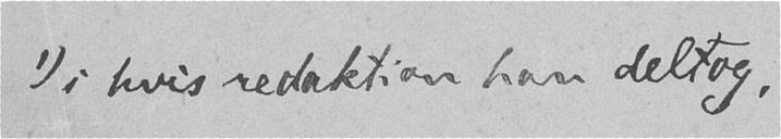1) i hvis redaktion han deltog.
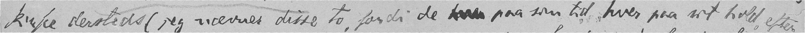kirke dersteds (jeg nævner disse to, fordi de  paa sin tid hver paa sit hold, efter
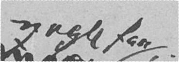nogle faa
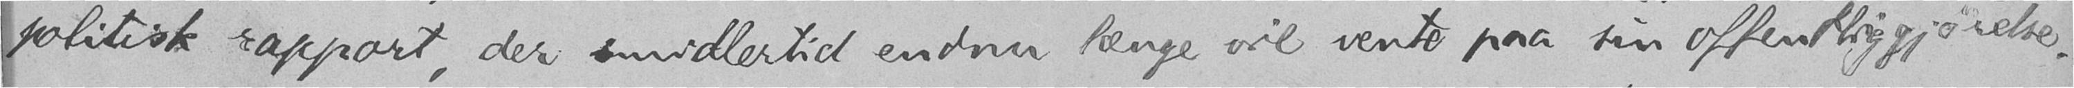politisk rapport, der imidlertid endnu længe vil vente paa sin offentliggjørelse.
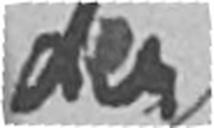der
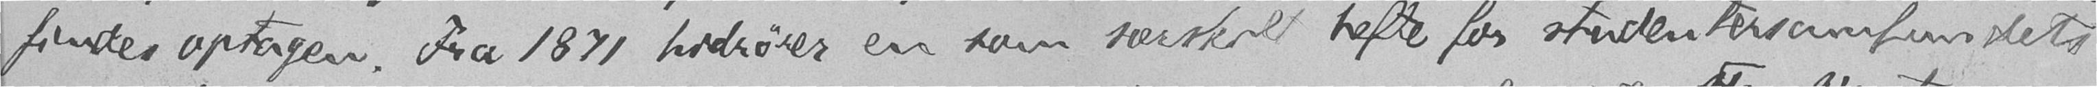findes optagen. Fra 1871 hidrører en som særskilt hefte for studentersamfundets
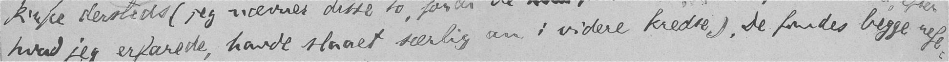hvad jeg erfarede, havde slaaet særlig an i videre kredse). De findes begge refe-
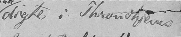digte i Throndhjems
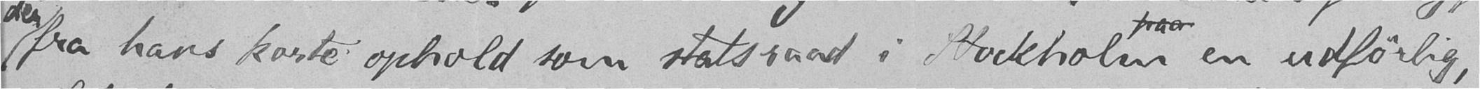fra hans korte ophold som statsraad i Stockholm en udførlig,
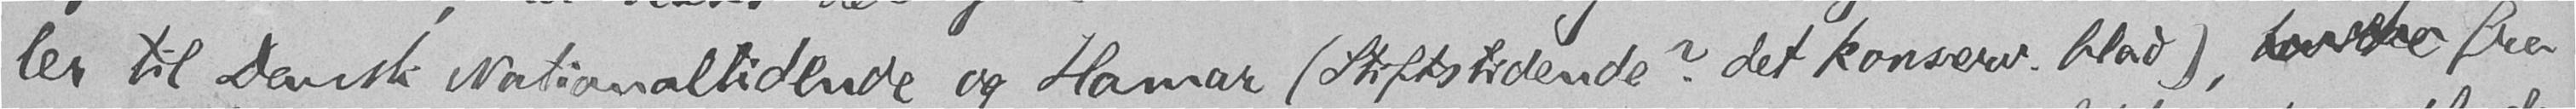ler til Dansk Nationaltidende og Hamar (Stiftstidende? det konserv. blad),  fra
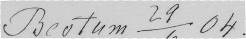Bestum 29/6 04
